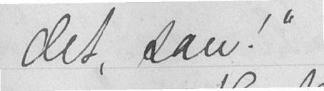det, san!"
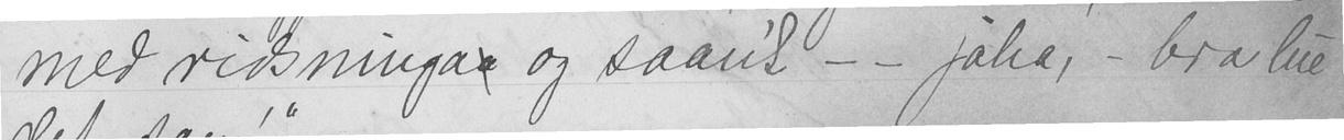med ridsningaa og saan't - - jaha, - bra lue
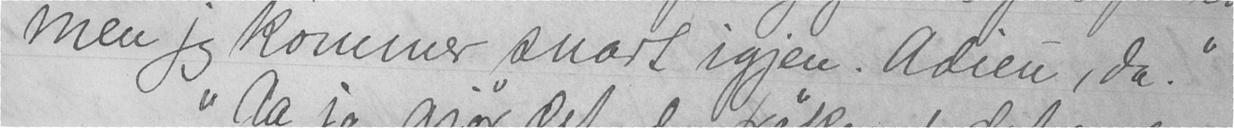men jeg kommer snart igjen. Adieu, da."
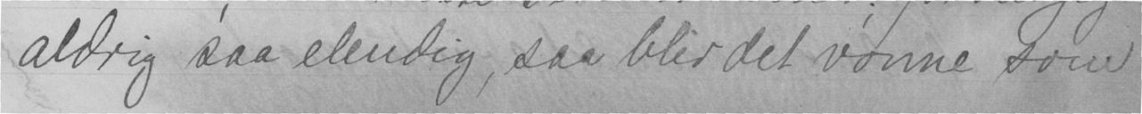aldrig saa elendig, saa blir det vonne som
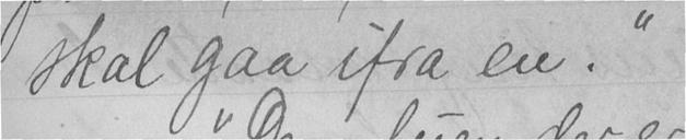skal gaa ifra en. "
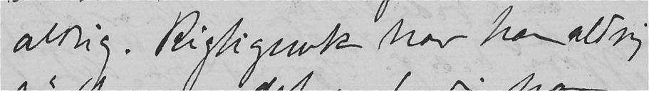aldrig. Rigtignok har han aldrig
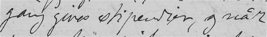gang gives stipender, og når
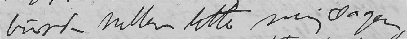burde heller lette mig sagen,
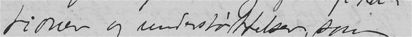tioner og understøttelser, som
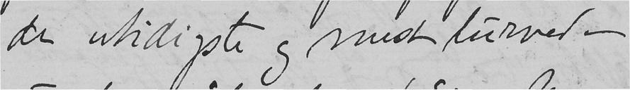de utidigste og mest lurvede
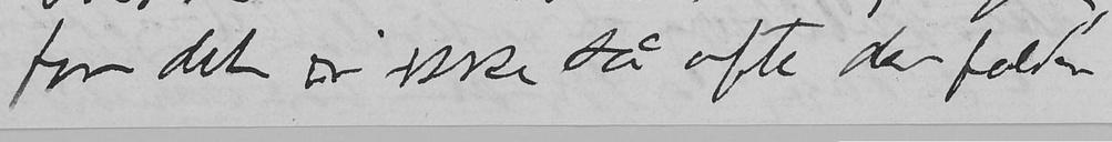for det er ikke så ofte der falder
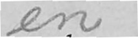en
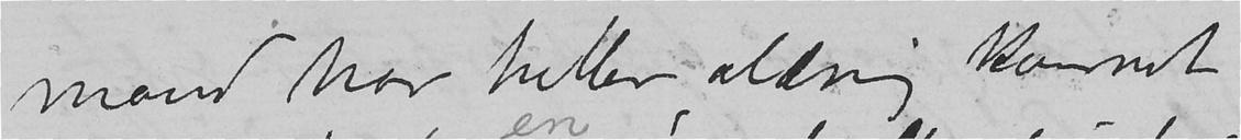mand har heller aldrig kunnet
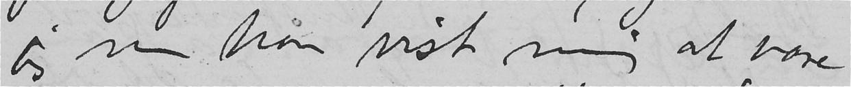jeg nu har vist meg at være
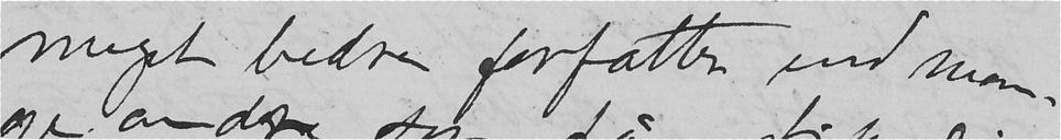meget bedre forfatter end man-
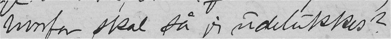hvorfor skal så jeg udelukkes?
